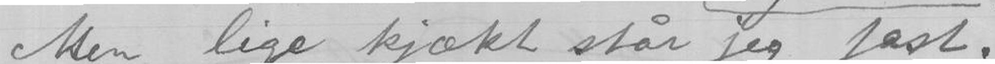Men lige kjækt står jeg fast.
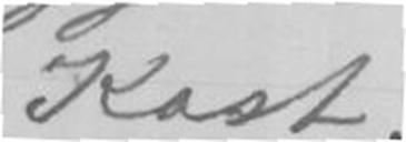Kast.
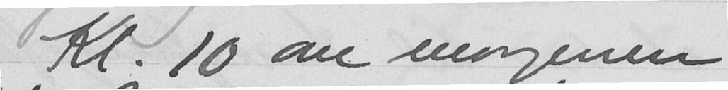Kl. 10 om morgenen
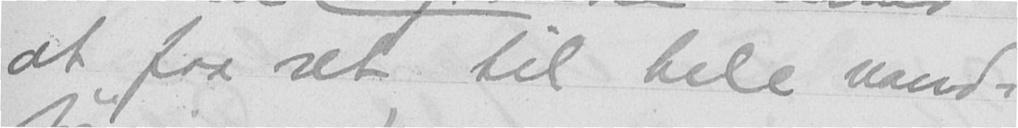at faa ret til hele vand-
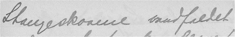Stangeskovene vandfaldet
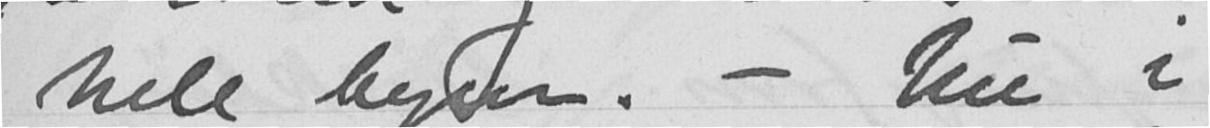hele byen. - Nu i
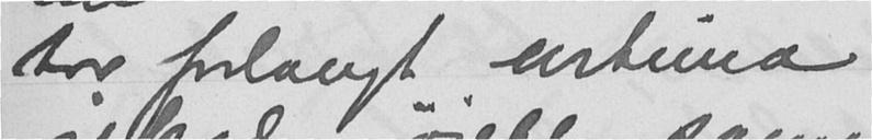har forlangt urtima
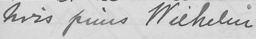hvis prins Wilhelm
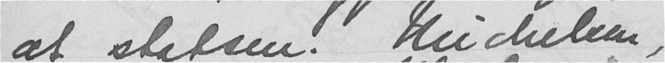at statsm. Michelsen,
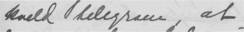kveld telegram, at
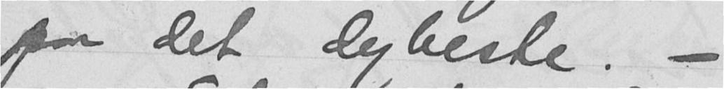paa det dybeste. -
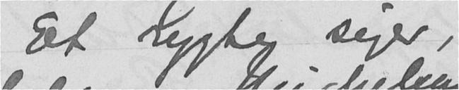Et rygte siger,
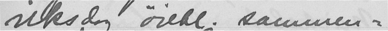riksdag øiebl. sammen-
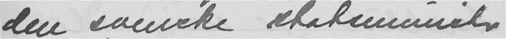den svenske statsminster
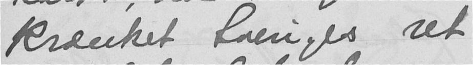krænket Sveriges ret
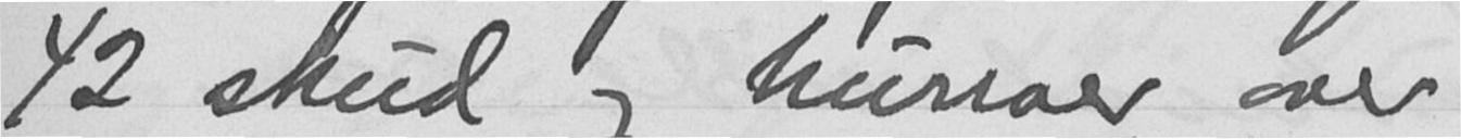42 skud og hurraer over
